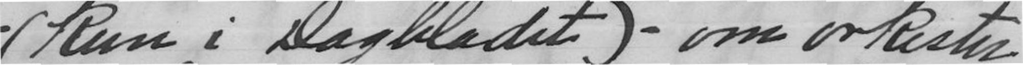-( kun i Dagbladet)- om orkester-
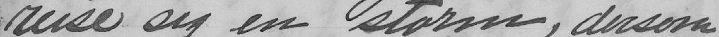reise sig en storm, dersom
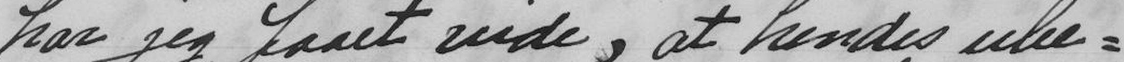har jeg faaet vide, at hendes ube-
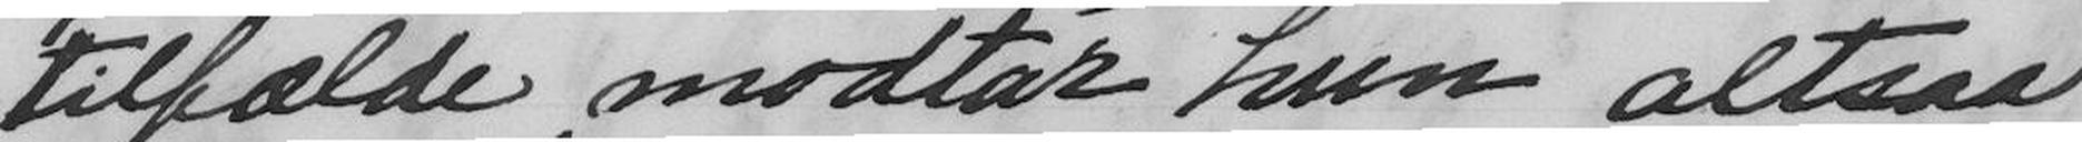tilfælde modtar hun altsaa
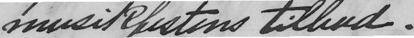musikfestens tilbud.
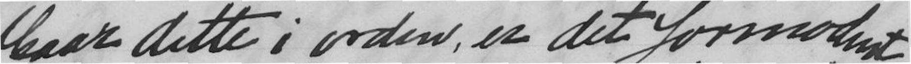Gaar dette i orden, er det formodent-
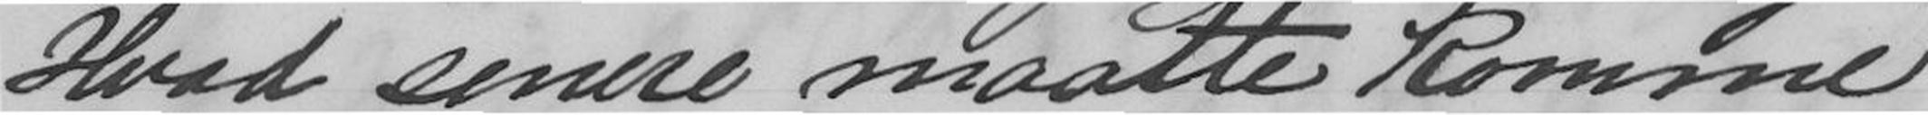Hvad senere maatte komme
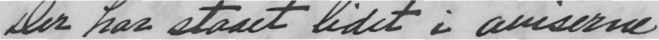Der har staaet lidet i aviserne
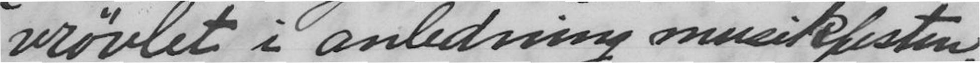vrøvlet i anledning musikfesten.
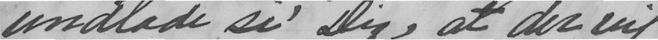undlade si' Dig, at der vil
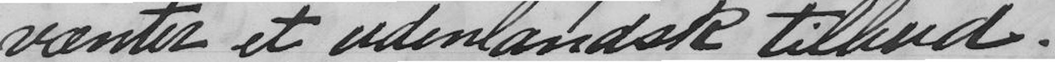vænter et udenlandsk tilbud.
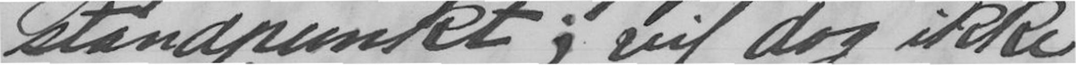standpunkt; vil dog ikke
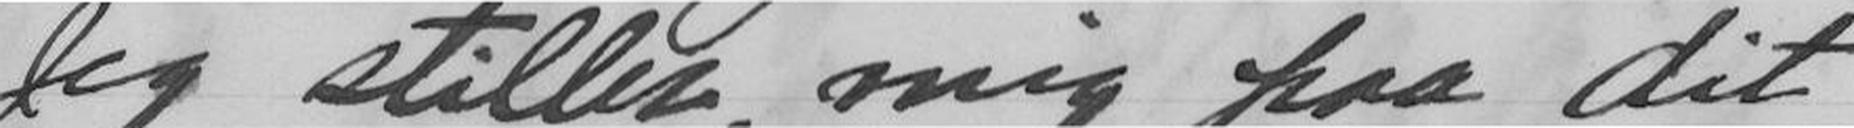Jeg stiller mig paa dit
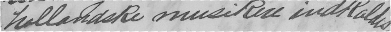hollandske musikere indkaldes
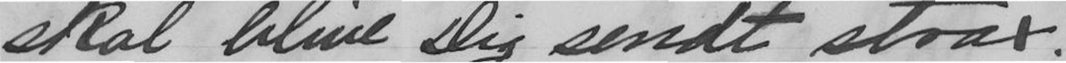skal blive Dig sendt strax.
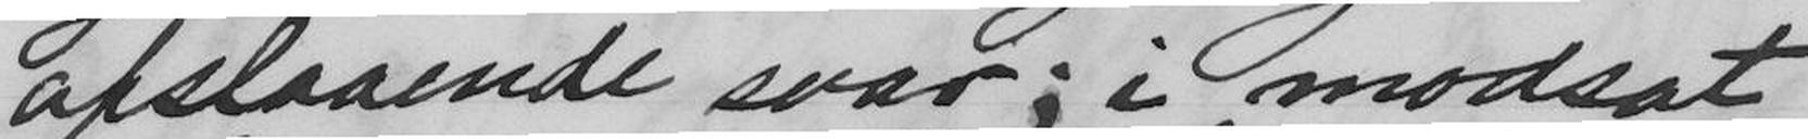afslaaende svar; i modsat
